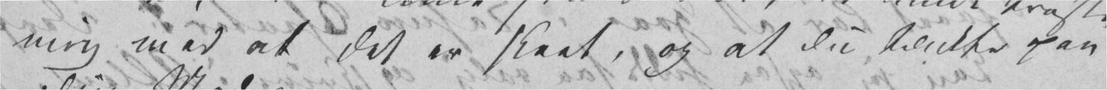mig med at det er skeet, og at Du tænkte paa
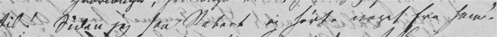til! Siden jeg saa Robert og hørte noget fra ham!
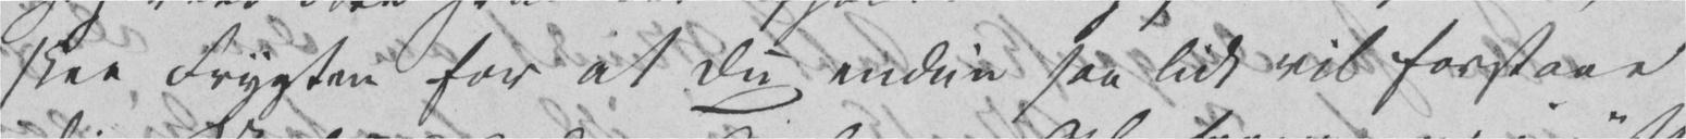skee Frygten for at Du endnu saa lidt vil forstaae
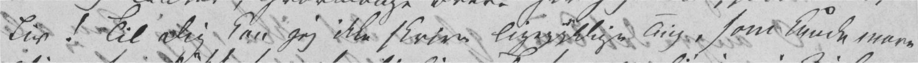Liv! Til Dig kan jeg ikke skrive ligegyldige Ting, som kunde more
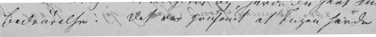Bedrøvelse. Det var grusomt at Ingen havde
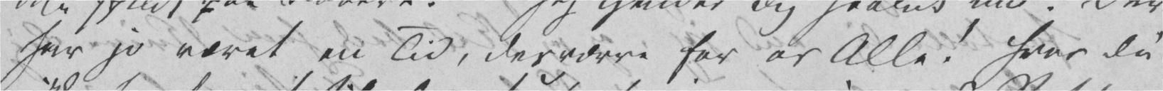har jo været en Tid, desværre for os Alle! hvor Du
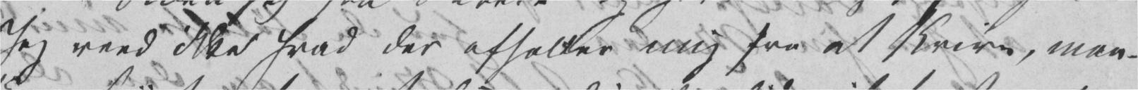Jeg veed ikke hvad der afholder mig fra at skrive, maa-
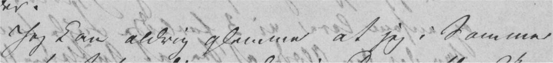Jeg kan aldrig glemme at jeg i Sommer
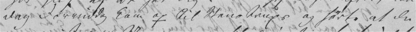dag Formiddag kom op til Stenstrups og hørte at Du
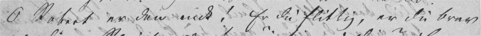O Robert er den endt! Er Du flittig, er Du brav
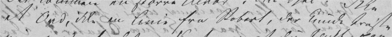et Ord, ikke en Linie fra Robert, der kunde trøste
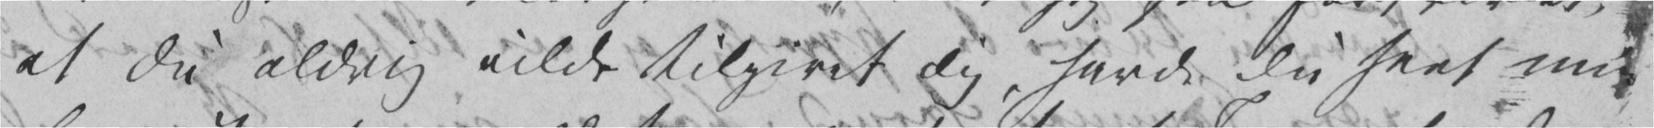at Du aldrig vilde tilgivet Dig, havde Du seet min
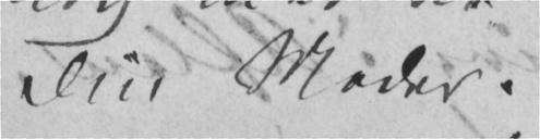Din Moder.
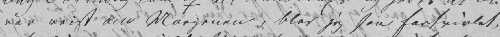var reist om Morgenen, blev jeg saa fortvivlet,
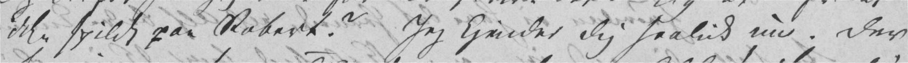ikke spildt paa Robert? Jeg kjender Dig saalidt nu. Der
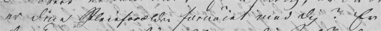er Dine Pleieforældre fornøiet med Dig? Er
